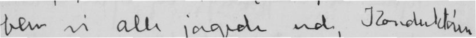blev vi alle jagede ud, Konduktøren
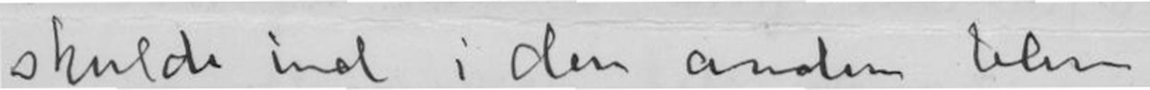skulde ind i den anden blive
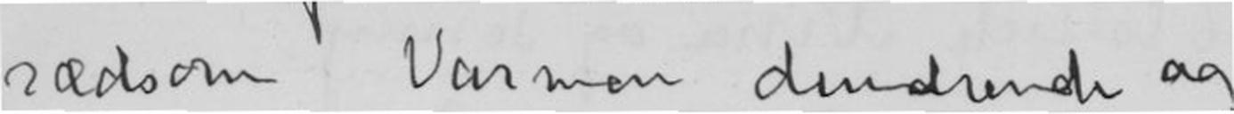rædsom, Varmen dundrende og
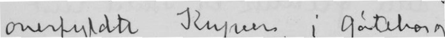overfyldte Kupeer, i Gøteborg
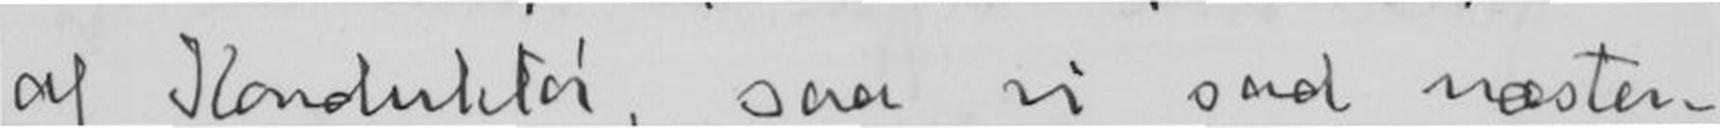af Konduktør, saa vi sad næsten
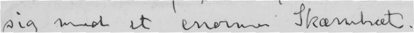sig med et enorm skærmbræt.
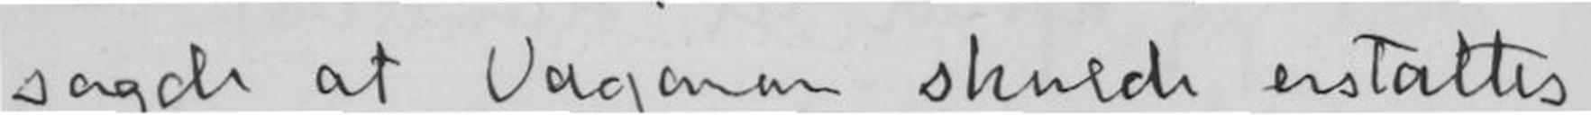sagde at Vagonen skulde erstattes
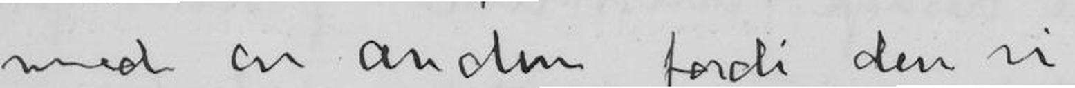med en anden fordi den vi
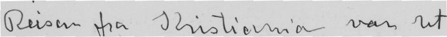Reisen fra Kristiania var ret
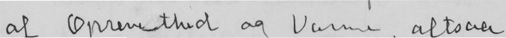af Oprenthed og Varme, altsaa
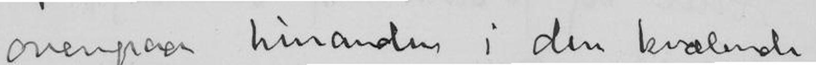ovenpaa hinanden i den kvælene
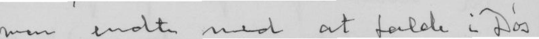men endte med at falde i Døs
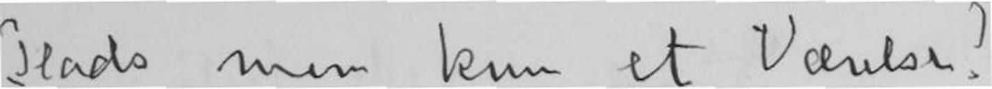Plads men kun et Værelse?
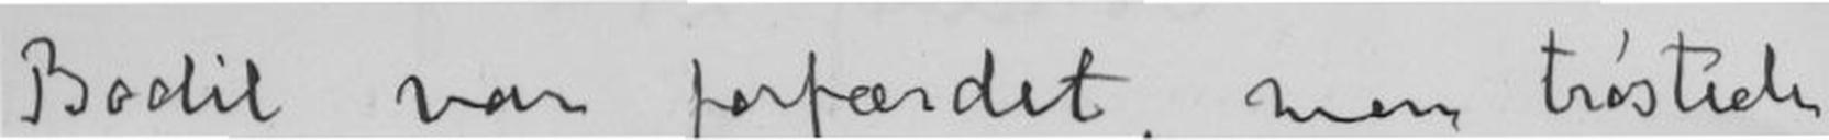Bodil var forfærdet, men trøstede
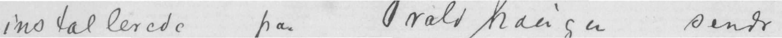installerede paa Troldhaugen sender
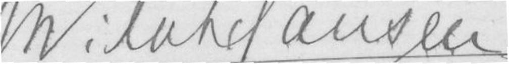Wilhelm Hansen
